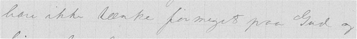bare ikke tænke for meget paa Gud og
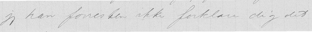jeg kan forresten ikke forklare dig det
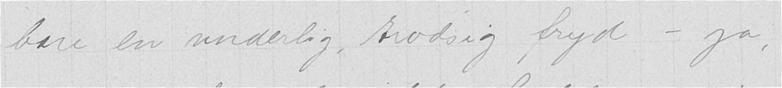bare en underlig, trodsig fryd - ja,
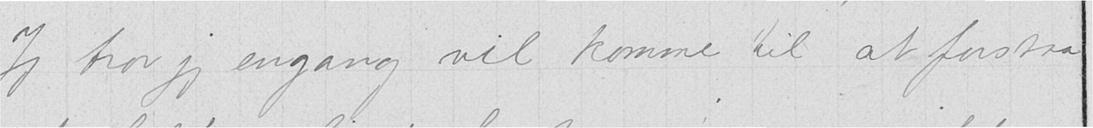Jeg tror jeg engang vil komme til at forstaa
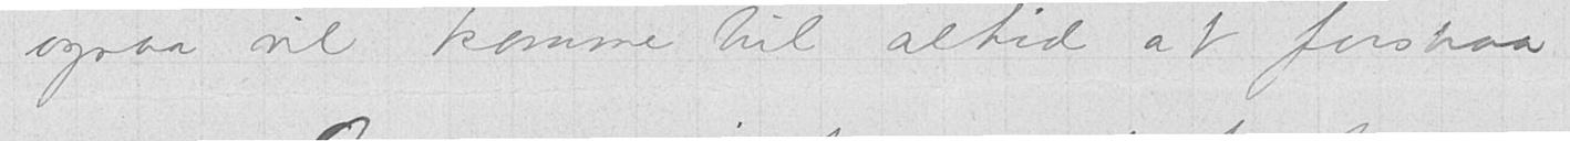ogsaa vil komme til altid at forstaa
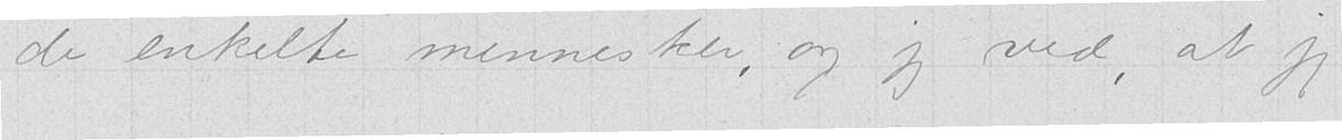de enkelte mennesker, og jeg ved, at jeg
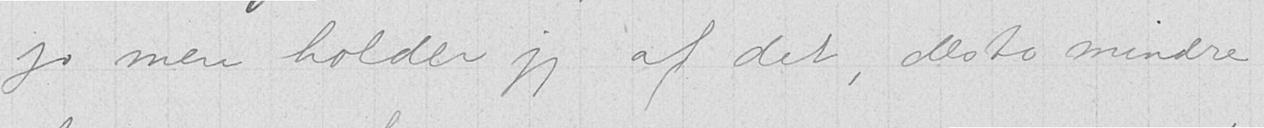jo mere holder jeg af det, desto mindre
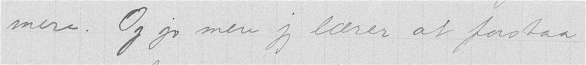mere. Og jo mere jeg lærer at forstaa,
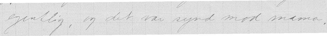egentlig, og det var synd mod mama,
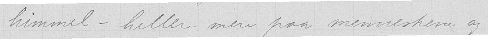himmel - hellere mere paa menneskene og
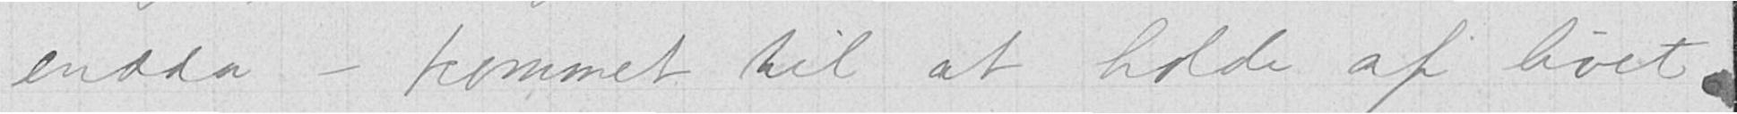endda - kommet til at holde af livet.
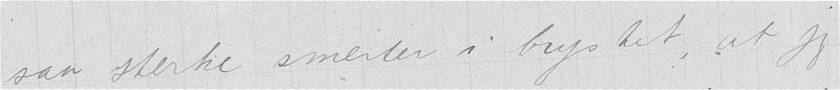saa sterke smerter i brystet, at jeg
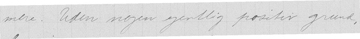mere. Uden nogen egentlig positiv grund,
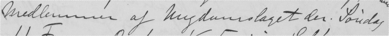Medlemmer af Ungdomslaget der. Søndag
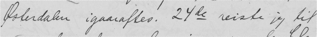Østerdalen igaaraftes. 24de reiste jeg til
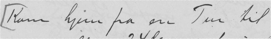Kom hjem fra en Tur til
